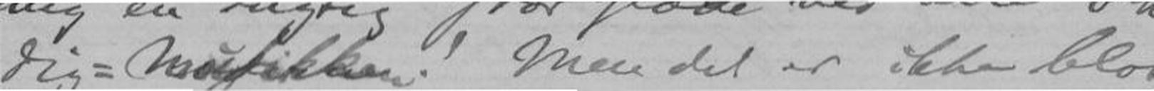dig-Musikken! Men det er ikke blot
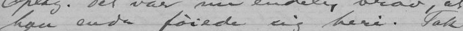han enda føiede sig heri. Tak
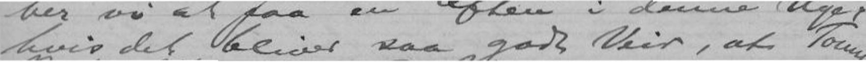hvis det bliver saa godt Veir, at Tonny,
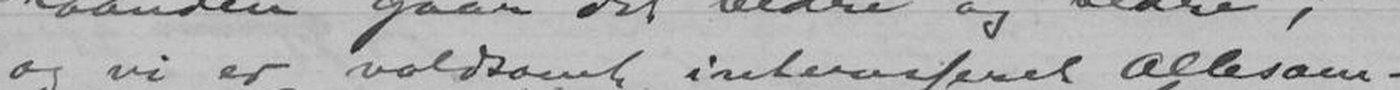og vi er voldsomt interesseret allesam-
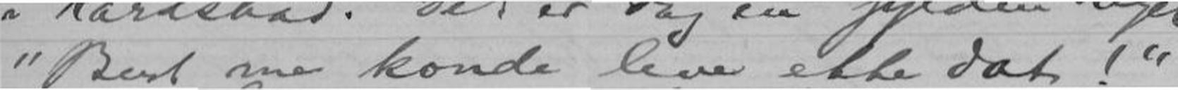"Best me konde leve ette dat!"
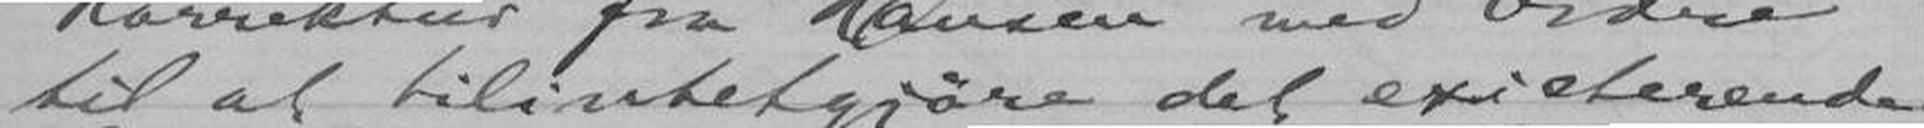til at tilintetgjøre det existerende
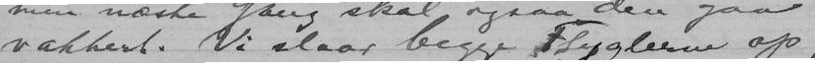vakkert. Vi slaar begge Flyglerne op,
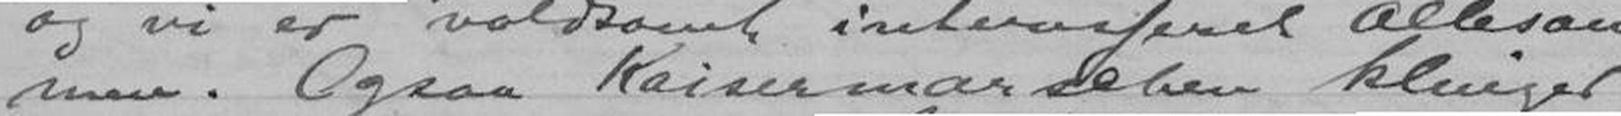en. Ogsaa Kaisermarschen klinger
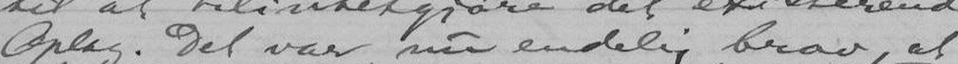Oplag. Det var nu endelig brad, at
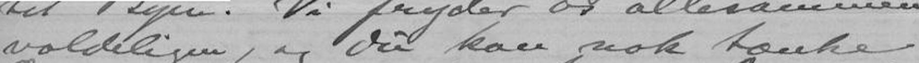voldeligen, og Du kan nok tænke
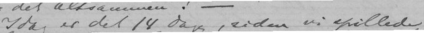Idag er det 14 Dage, siden vi spillede
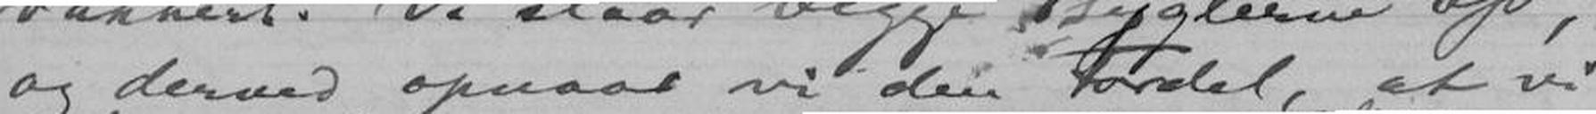og derved opnaar vi den Fordel, at vi
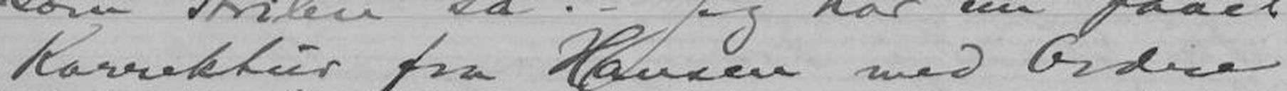Korrektur fra Hansen med Ordre
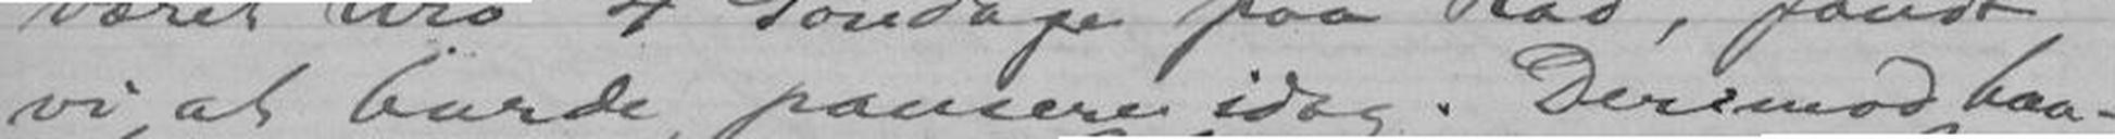vi at burde pausere idag. Derimod haa-
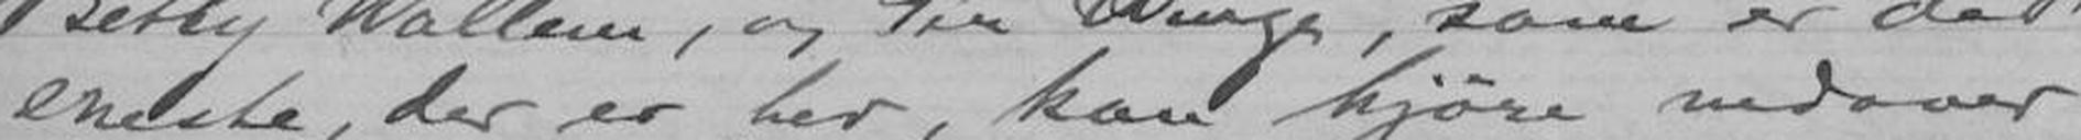eneste, der er her, kan kjøre nedover
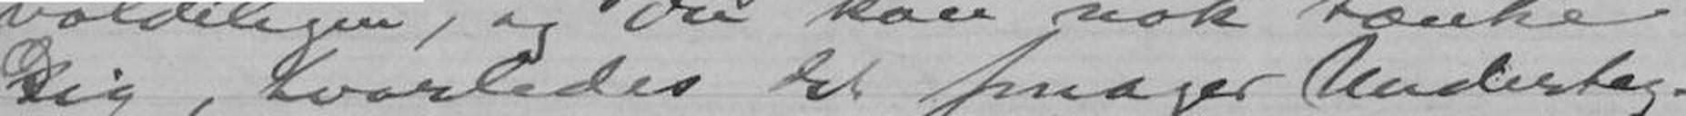Dig, hvorledes det smager Underteg-
